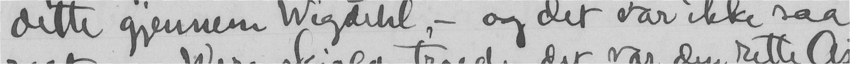dette gjennem Wigdehl, - og det var ikke saa
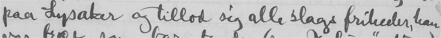paa Lysaker og tillod sig alleslags friheder, han
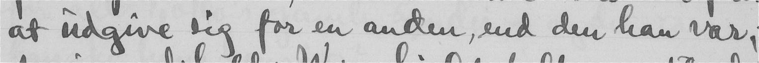at udgive sig for en anden, end den han var;
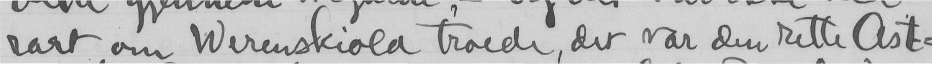rart om Werenskiold troede, det var den rette Ast-
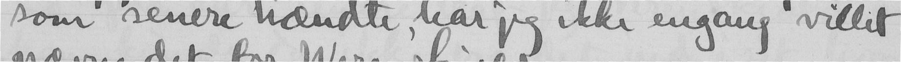som senere hændte, har jeg ikke engang villet
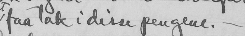faa tak i disse pengene.
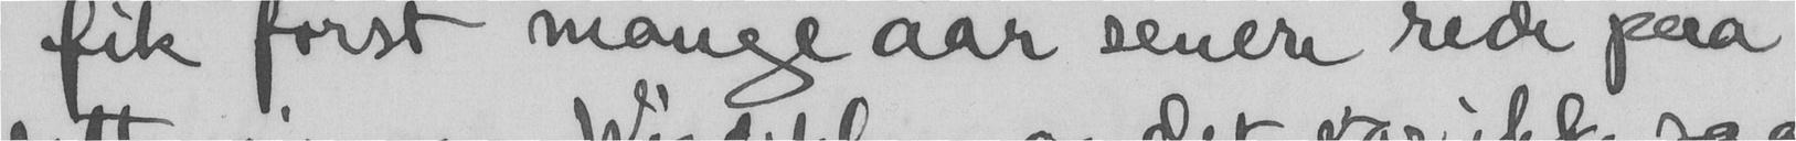fik først mange aar senere rede paa
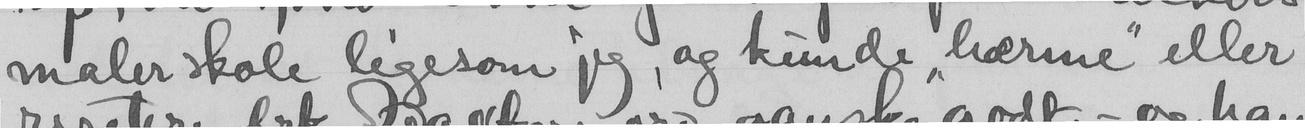malerskole ligesom jeg, og kunde "hærme" eller
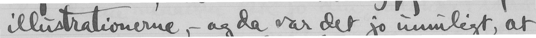illustrationerne, - og da var det jo umuligt, at
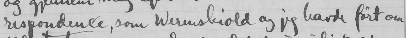respondence, som Werenskiold og jeg havde ført om
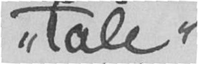"tale"
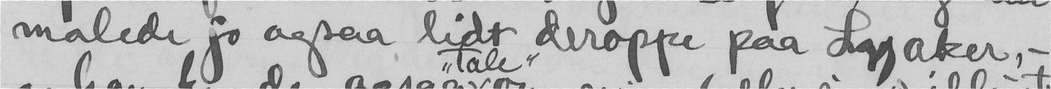malede jo ogsaa lidt deroppe paa Lysaker,-
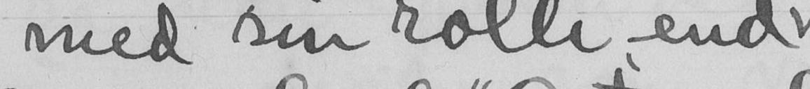med sin rolle end
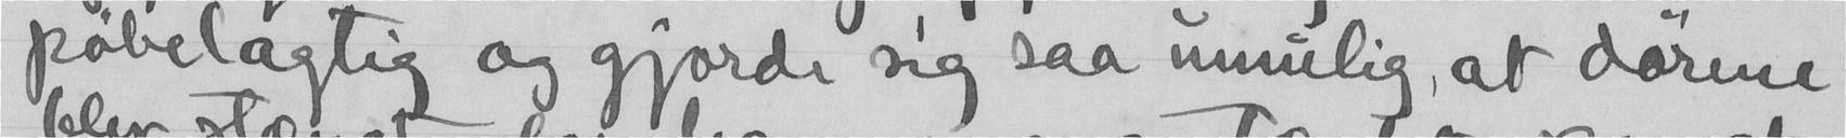pøbelagtig og gjorde sig saa umulig, at dørene
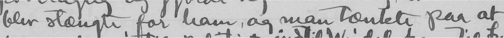blev stængte for ham, og man tænkte paa at
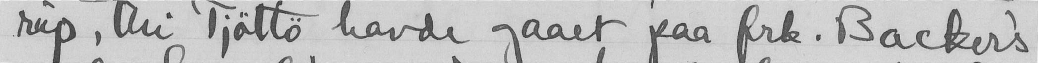rup, thi Tjøttø havde gaaet paa frk. Backers
